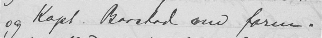og Kapt. Baastad om form.
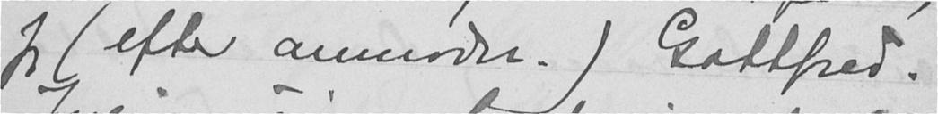jeg (efter anmodn.) Gottfred.
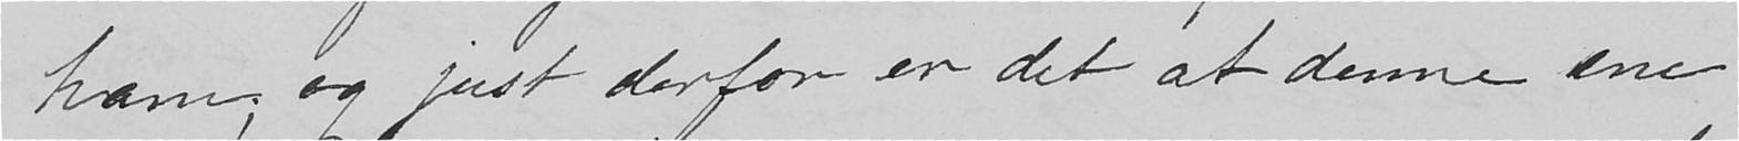ham, og just derfor er det at denne ene
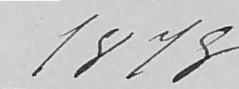1878
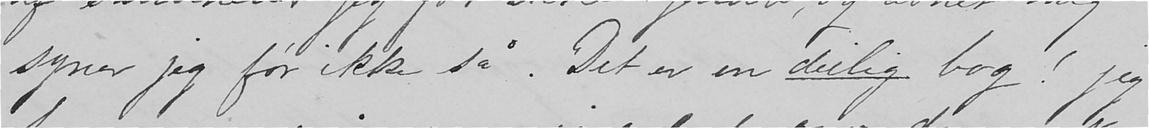syner jeg før ikke så. Det er en deilig bog! jeg
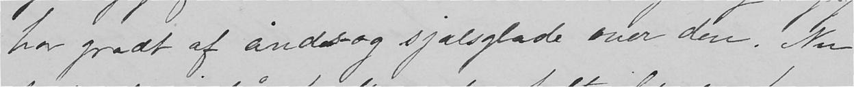har grædt af ånds- og sjælsglæde over den. Nu
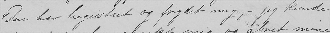Den har begeistret og frydet mig, - jeg kunde
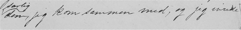dem, jeg kom sammen med, og jeg ivrede
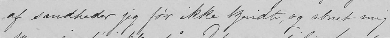af sandheder jeg før ikke kjendte, og åbnet mig
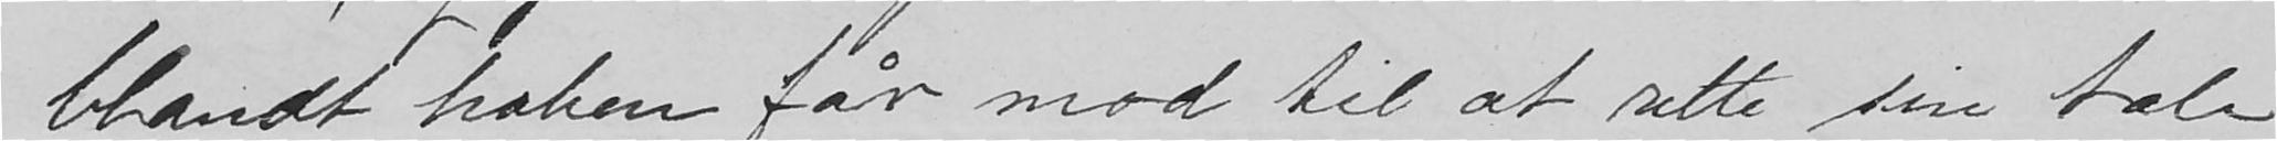blandt hoben får mod til at rette sin tale
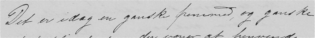Det er idag en ganske fremmed, og ganske
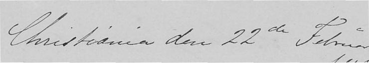Christiania den 22de Februar
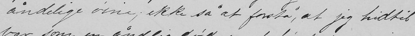åndelige øine; ikke så at forstå, at jeg hidtil
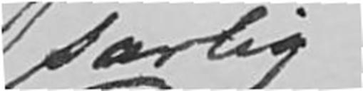særlig
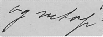og netop
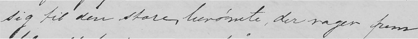sig til den store, berømte der rager frem
